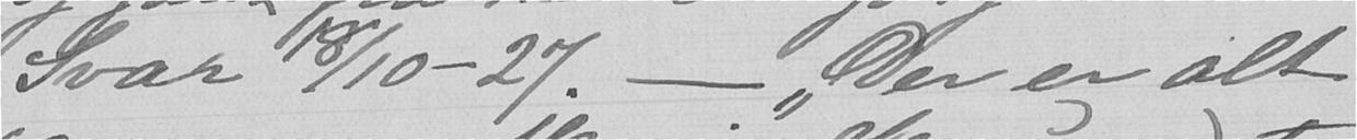Svar 18/10-27. - "Der er alt
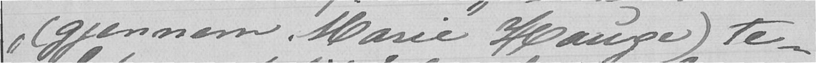"(gjennem Marie Hauge) te-
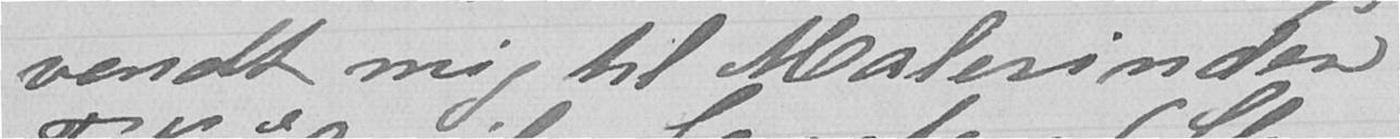vendt mig til Malerinden
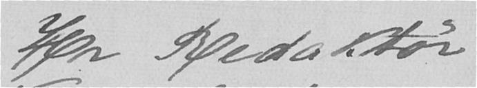Hr Redaktør
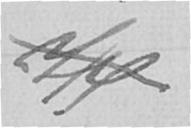H
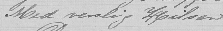Med venlig Hilsen
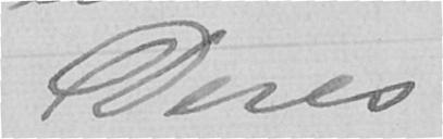Deres
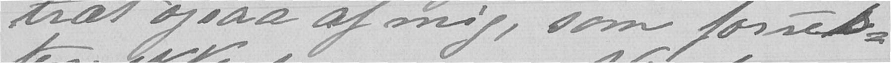træt ogsaa af mig, som forres-
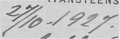27/10-1927.
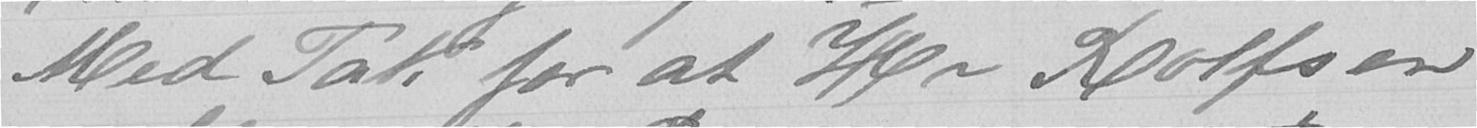Med Tak for at Hr Rolfsen
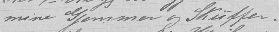mine Gjemmer og Skuffer.
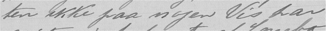ten ikke paa nogen Vis har
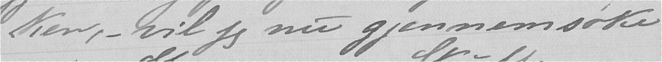ken, - vil jeg nu gjennemsøke
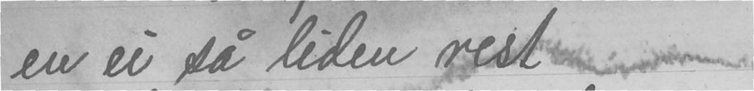en ei så liden rest
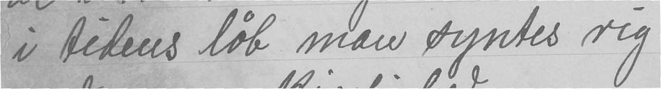i tidens løb man syntes rig
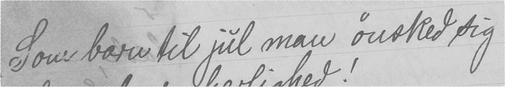Som barn til jul man ønsked sig
